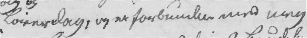Løverdag, og er forbunden med meg
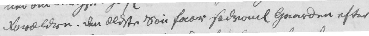Forældre. Den ældste Søn faar sædvanl Gaarden efter
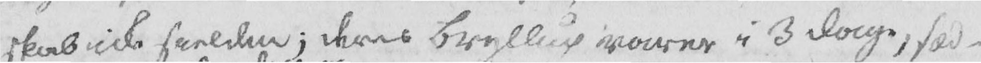skab icke sielden; deres Bryllup varer i 3 Dage, sæd-
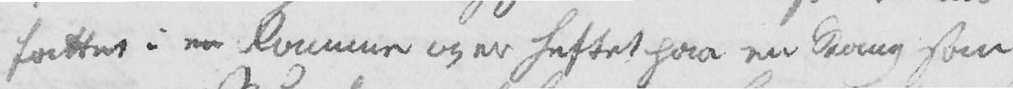fattet i en Ramme og er festet paa en Stang som
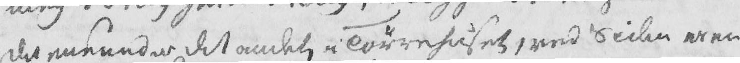det ene under det andet i Tørrehuset, ved Siden er en
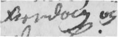Fredag og
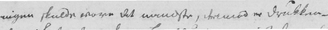ingen skulde vove det mindste, derimod er Drukken-
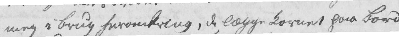meg i brug heromkring, de lægge Kornet paa Bord
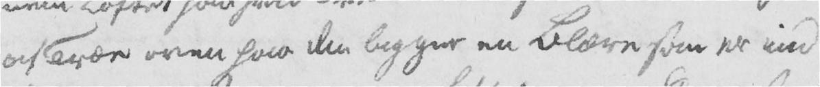af Træe over paa den ligger en Blære som er ind
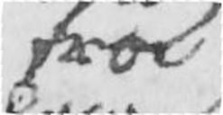fra
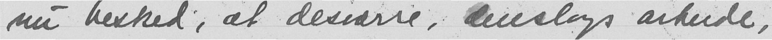nu besked, at desværre, denslags arbeide,
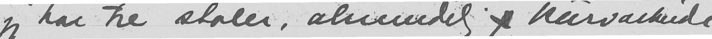jeg har tre stoler, almindelig  kurvarbeide
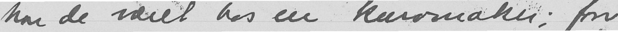har de været hos en kurvmaker; faar
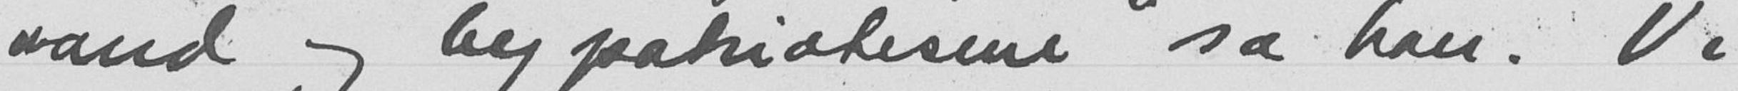vand og bypatriotisme” sa han. Vi
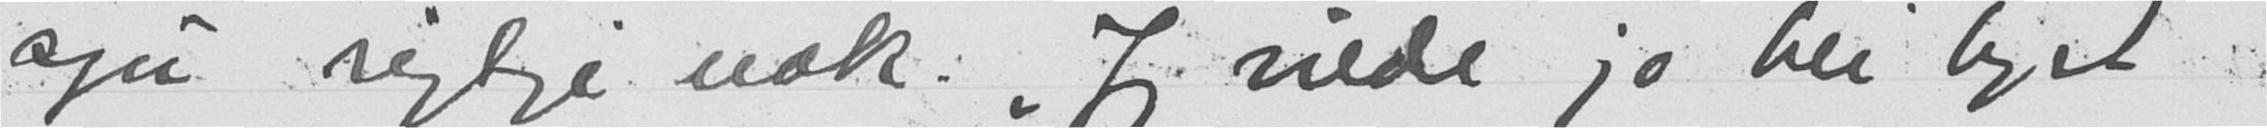sgu rigtige nok. „Jeg vilde jo bli lyst
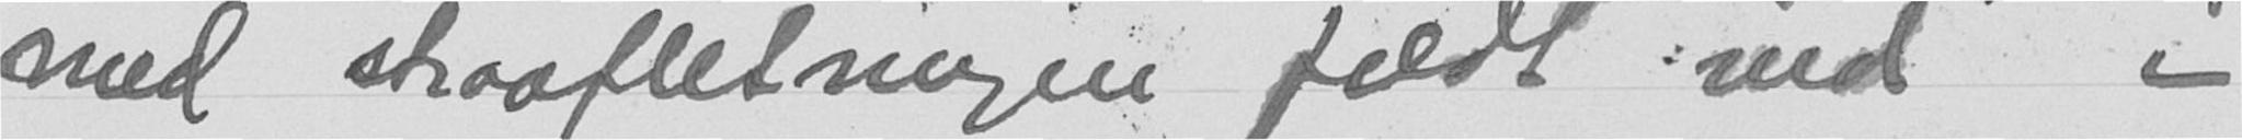med straafletningen faldt ind i
